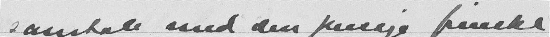samtale med den forrige finske
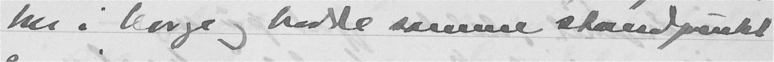her i Norge og hadde samme standpunkt
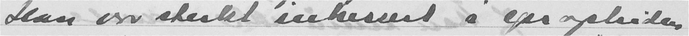Han var spesielt interessert i sprogstriden
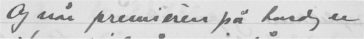Og når premieren på torsdag er
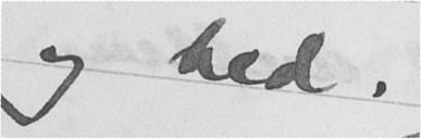og bed.
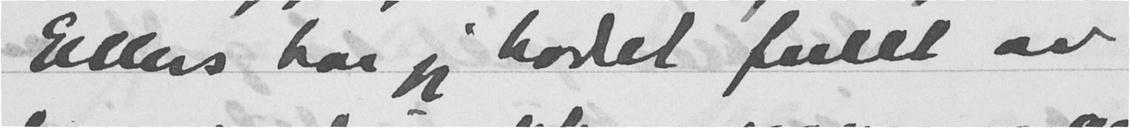Ellers har jeg hodet fullt av
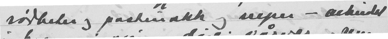rødbeter og pastinakk og neper - arbeidet
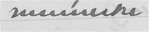menneske -
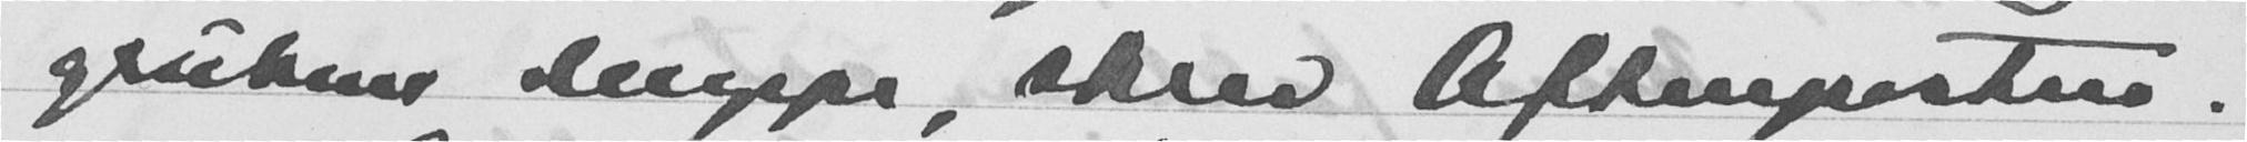gruben deroppe, skrev Aftenposten.
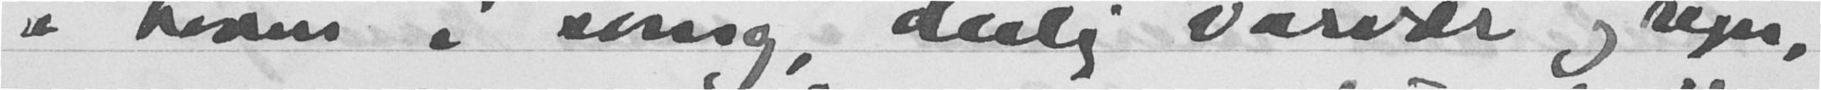i haven i sving, deilig vårvær og regn,
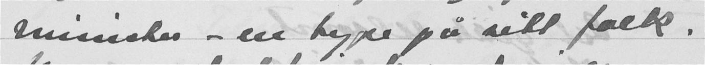minster - en type på sitt folk.
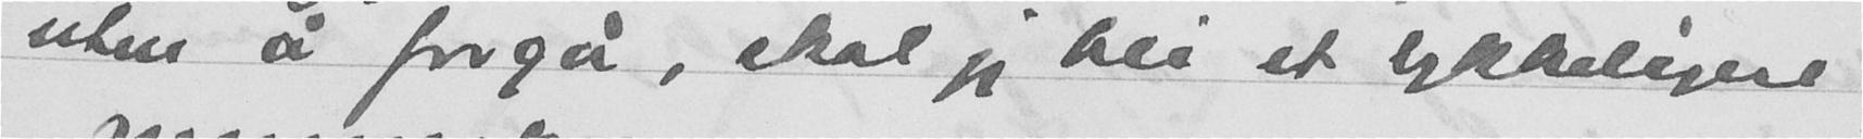uten å forgå, skal jeg bli et lykkeligere
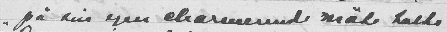"på sin egen charmerende måte talte
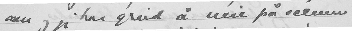over og vi har greid å neie på scenen
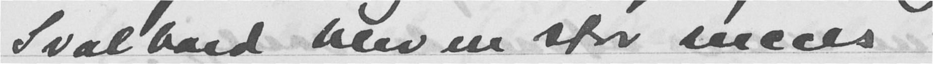Svalbard blev en stor succes
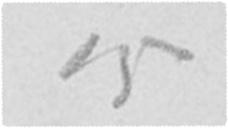15
